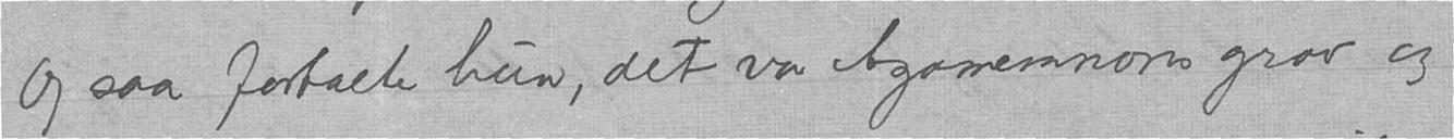Og saa fortalte hun, det var Agamemnons grav og
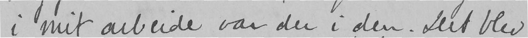i mit arbeide var der i den. Det blev
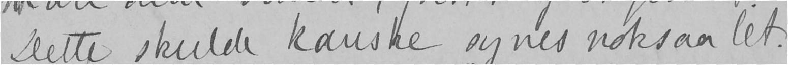Dette skulde kanske synes noksaa let.
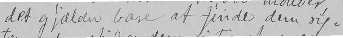det gjælder bare at finde dem rig-
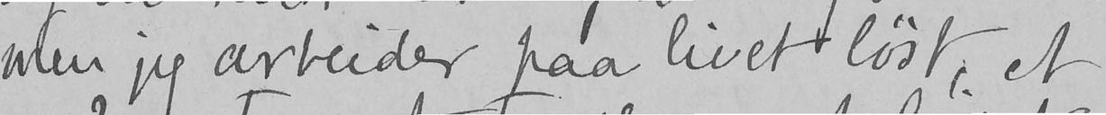men jeg arbeider paa livet løst, et
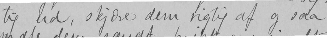tig ud, skjære dem rigtig af og saa
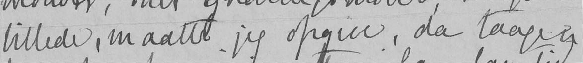billede, maatte jeg opgive, da taagen
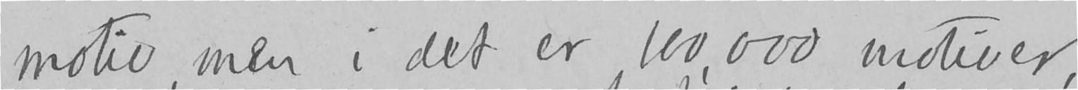motiv, men i det er 100,000 motiver,
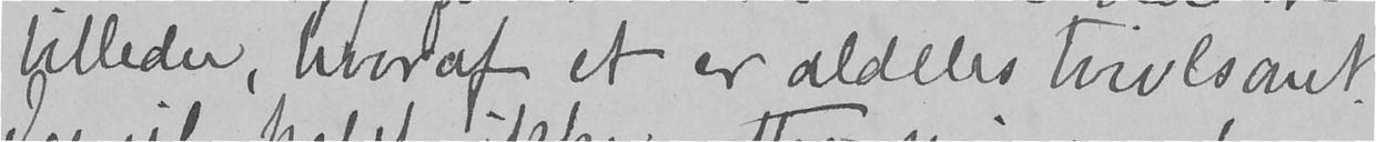billeder, hvoraf et er aldeles tvivlsomt.
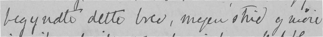begyndte dette brev, megen strid og møie
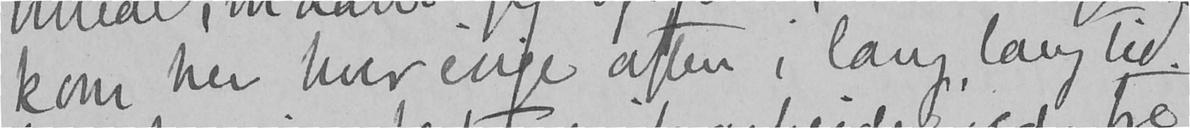kom her hver evige aften i lang, lang tid.
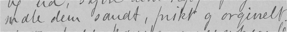male dem sandt, friskt og orginelt.
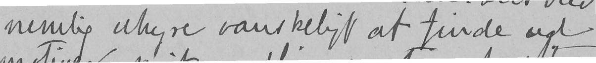nemlig uhyre vanskeligt at finde ud
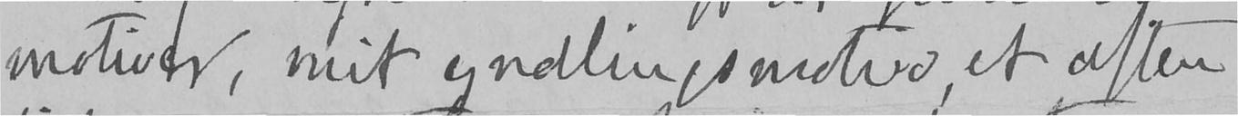motiver, mit yndlingsmotiv, et aften
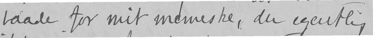baade for mit menneske, der egentlig
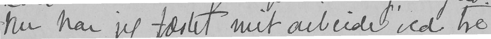Nu har jeg fæstet mit arbeide ved tre
